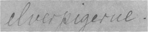elverpigerne.
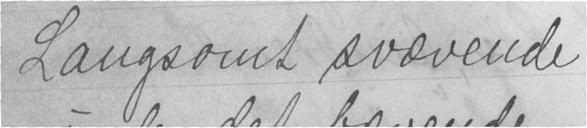Langsomt svævende
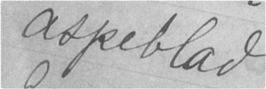aspeblad
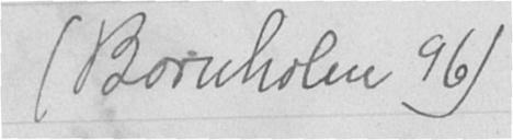(Bornholm 96)
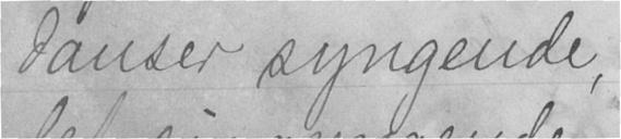danser syngende,
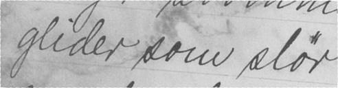glider som slør
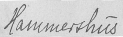Hammershus
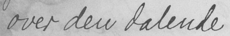over den dalende
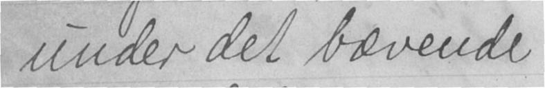under det bævende
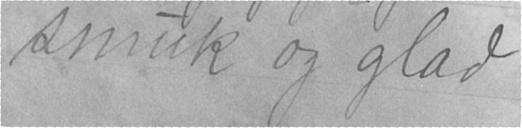smuk og glad
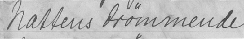Nattens drømmende
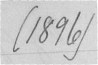(1896)
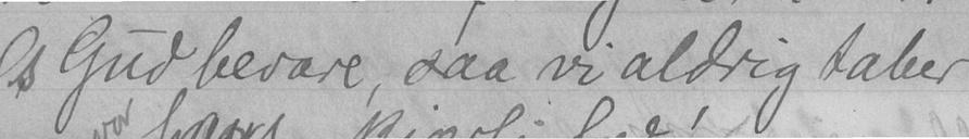Os Gud bevare, saa vi aldrig taber
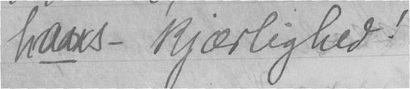hans- kjærlighed!
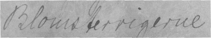Blomsterrigerne
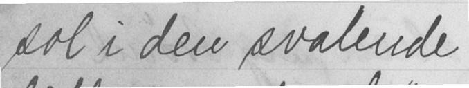sol i den svalende
